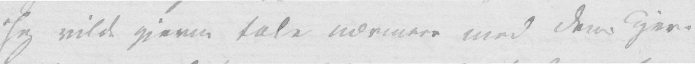Jeg vilde gjerne tale nærmere med Dem kjere
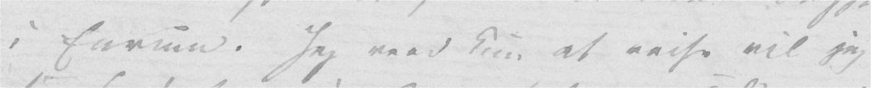i Enrum. Jeg veed kun at reise vil jeg
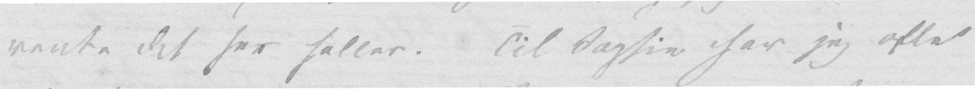vente det her heller. Til Sophie har jeg ofte
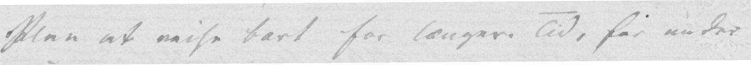Plan at reise bort for længere Tid, for under
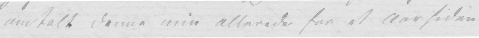omtalt denne min allerede for et Aar siden
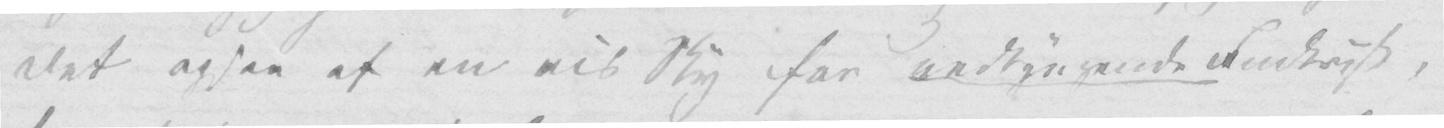det ogsaa af en vis Sky for nedtyngende Indtryk,
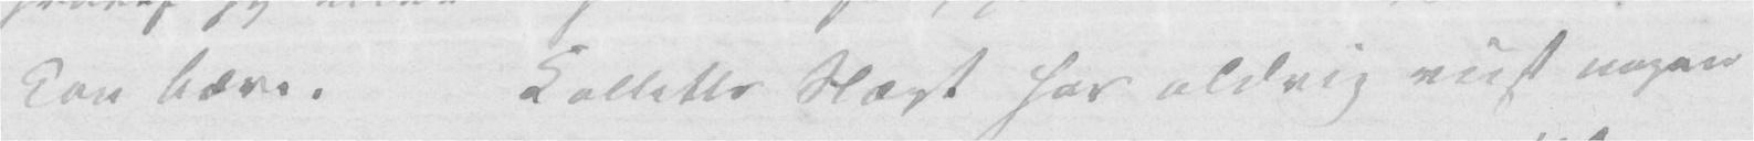kan bære. Colletts Slægt har aldrig viist nogen
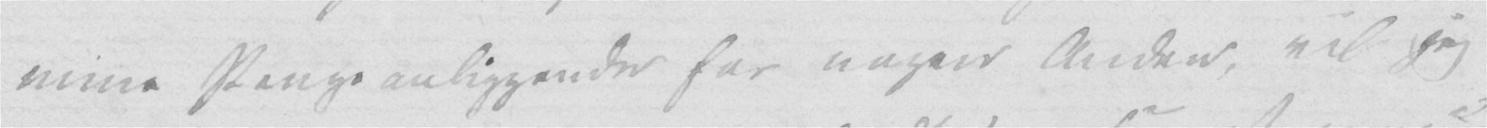mine Pengeanliggender for nogen Anden, vil jeg
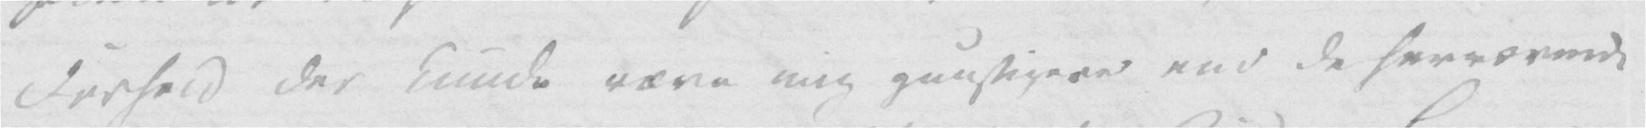Forhold der kunde være mig gunstigere end de herværende
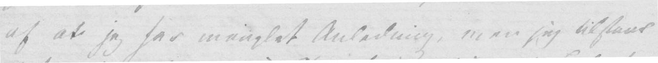af at jeg har manglet Anledning, men jeg tilstaar
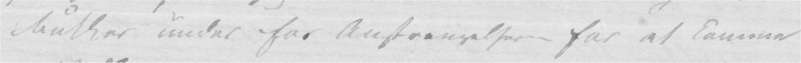bukker under for Anstrengelserne for at komme
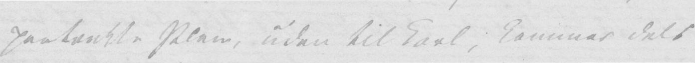paatænkte Plan, uden til Carl, kommer dels
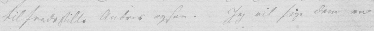tilfredsstille Andres ogsaa. Jeg vil sige Dem en
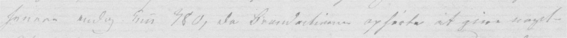senere endog kun 380, da Brandactierne ophørte at give noget –
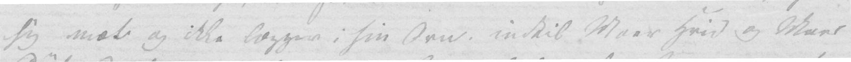sig mæt og ikke lægger i sin Ovn, indtil Moer Hvid og Moer
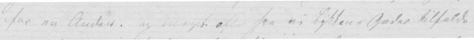for en Anden. og meget ofte see vi Lykkens Goder tilfalde
